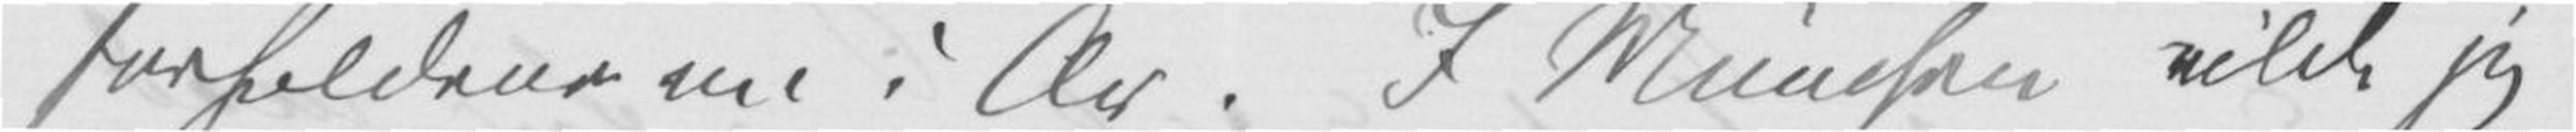forholdene nu i År. I München vilde jeg
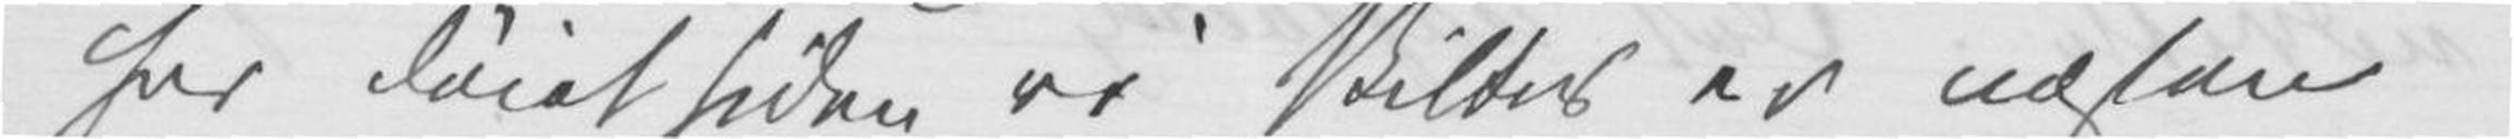har døiet siden vi skiltes er næsten
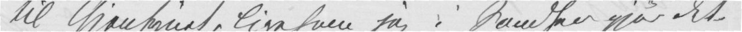til Gjensynet, ligesom jeg i Sandhed gjør det.
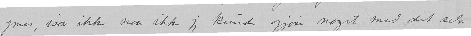pris, især ikke naar ikke jeg kunde gjøre noget med det selv
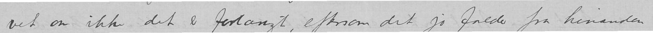vet om ikke det er forlangt, eftersom det jo falder fra hinanden
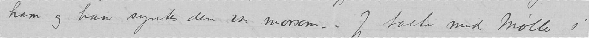ham og han syntes den var morsom. – Jeg talte med Møller i
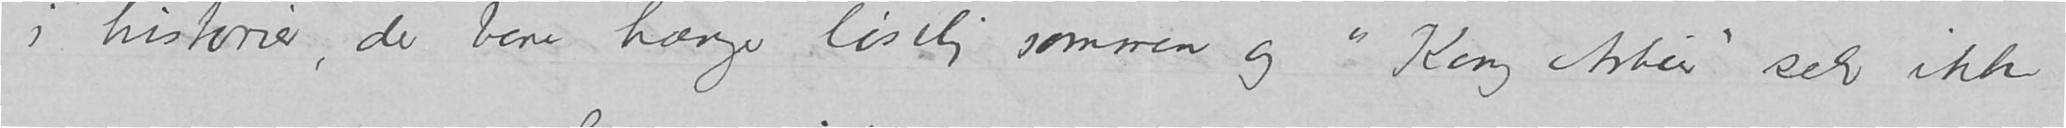i historier, der bare hænger løselig sammen og «Kong Artur» selv ikke
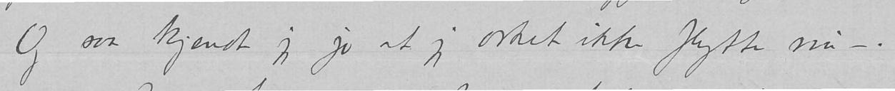Og saa kjendte jeg jo at jeg orket ikke flytte nu –.
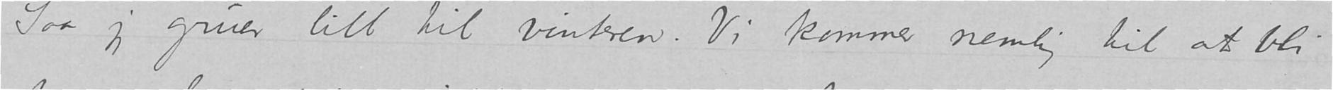Saa jeg gruer litt til vinteren. Vi kommer nemlig til at bli
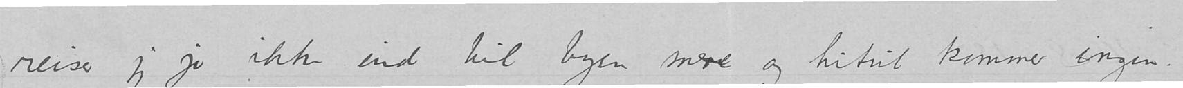reiser jeg jo ikke ind til byen mere og hitut kommer ingen.
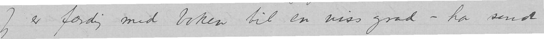Jeg er færdig med boken til en viss grad – har sendt
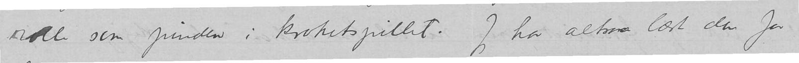rolle som pinden i kroketspillet. Jeg har altsaa læst den for
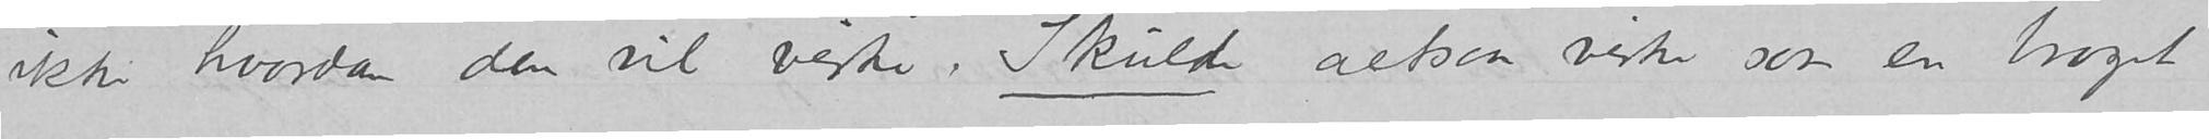ikke hvordan den vil virke. Skulde altsaa virke som en broget
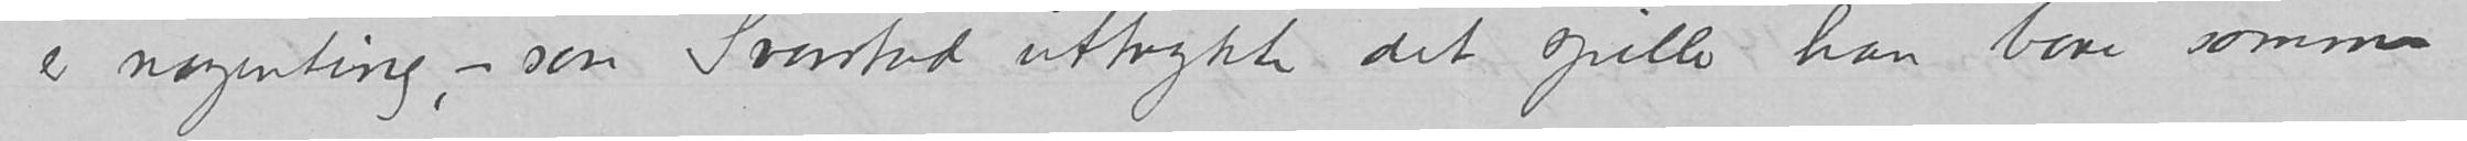er nogenting – som Svarstad uttrykte det spille han bare samme
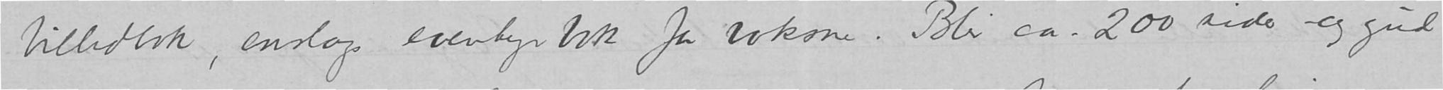billedbok, enslags eventyrbok for voksne. Blir ca. 200 sider – og gud
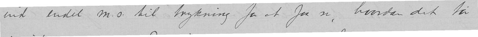ind endel m.s. til trykning for at faa se, hvordan det tar
